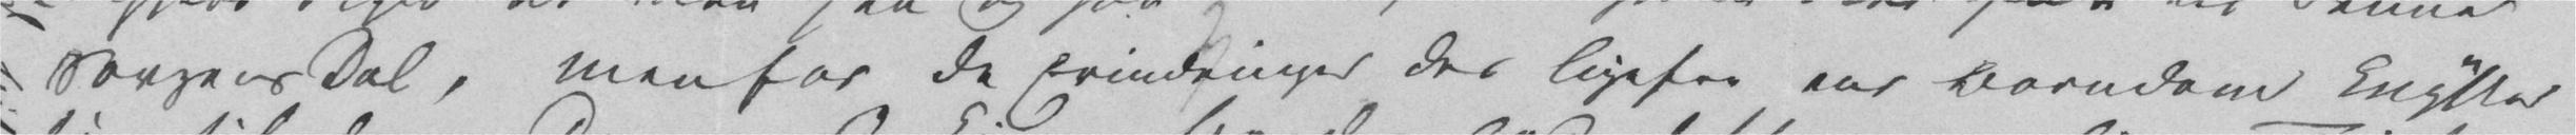Sorgens Dal, men for de Erindringer der ligefra vor Barndom knytter
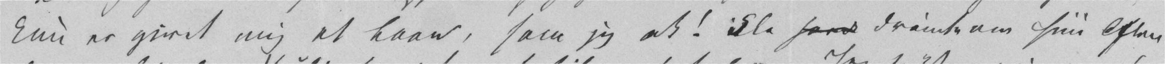kun er givet mig et Laan, som jeg ak! ikke havde drømte om hiin Aften
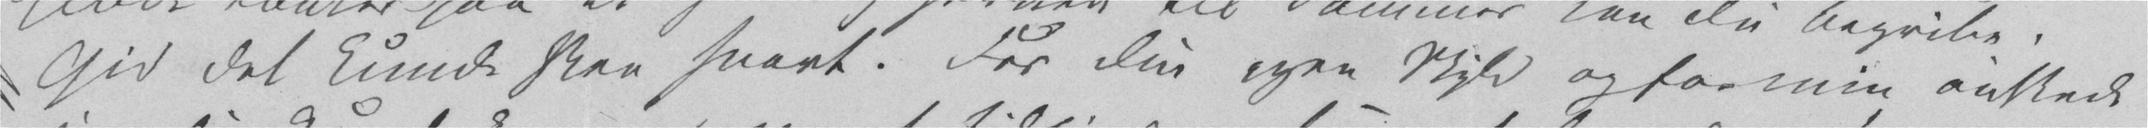Gid det kunde skee snart. For Din egen Skyld og for min ønskede
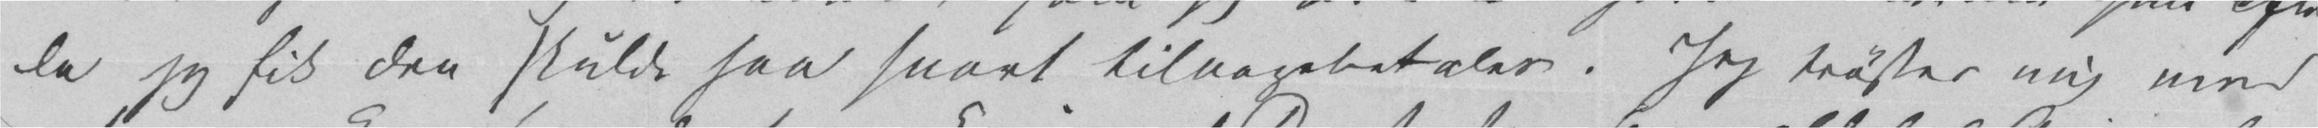da jeg fik den skulde saa snart tilbagebetales. Jeg trøster mig med
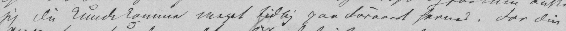jeg Du kunde komme meget tidlig paa Foraaret herned. For Din
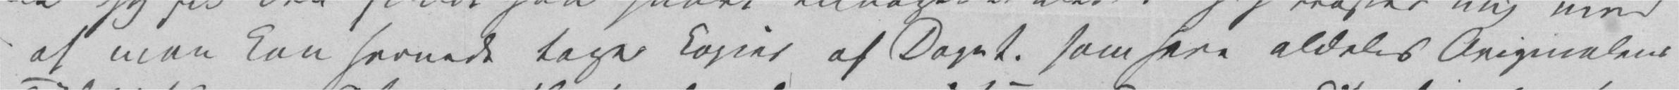at man kan hernede tage Kopier af Daget. som have aldeles Originalens
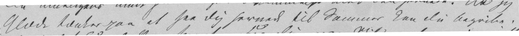Glæde tænker paa at see Dig hernede til Sommer kan Du begribe,
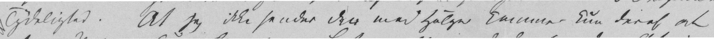Tydelighed. At jeg ikke sender den med Holger kommer kun deraf at
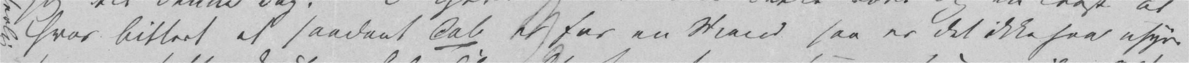hvor bittert et saadant Tab er for en Mand saa er det ikke saa uhyre
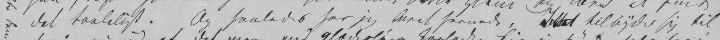det taaleligt. Og saalede har jeg levet hernede, Intet tilbyder sig til
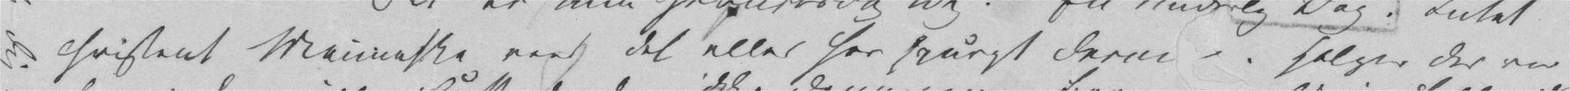christent Menneske veed det eller har spurgt derom. Holger der var
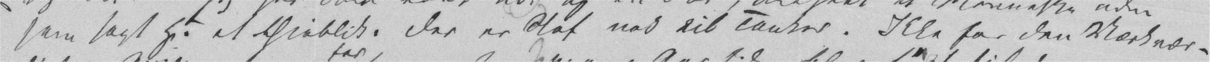som sagt H. et Øieblik. Der er Stof nok til Tanker. Ikke for den Mærkvær
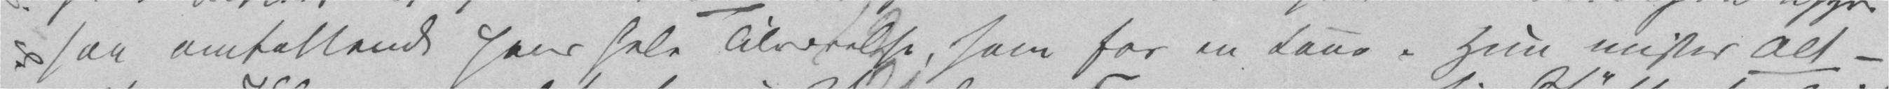saa omfattende hans hele Tilværelse, som for en Kone. Hun mister Alt –
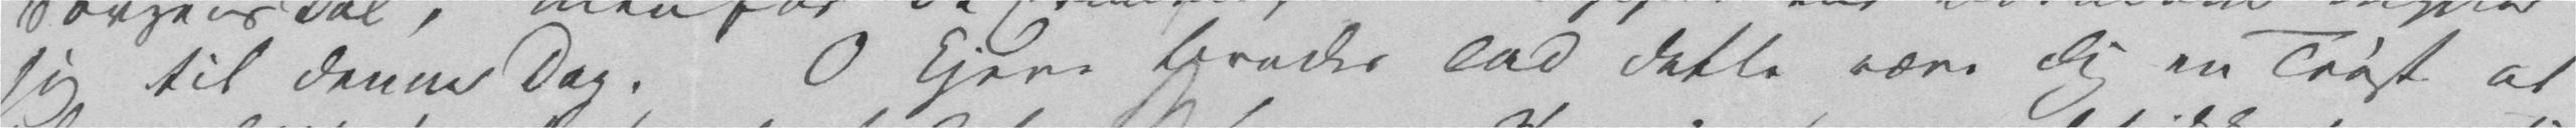sig til denne Dag. O kjere Broder lad dette være Dig en Trøst at
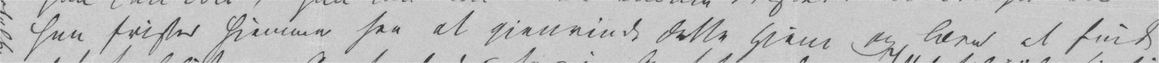hun frister hjemme see at gjenvinde dette Hjem og lære at finde
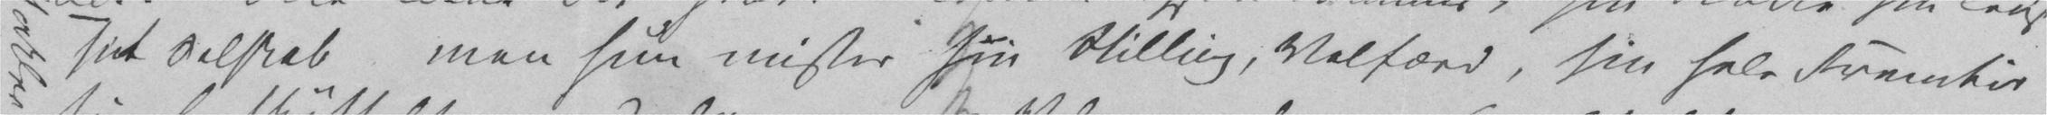sit Selskab men hun mister sin Stilling, Velfærd, sin hele Fremtid
